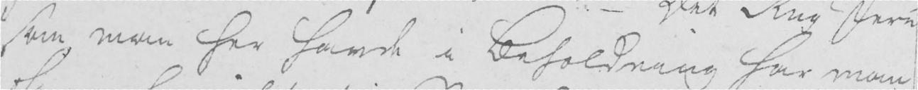som man her havde i Beholdning har man
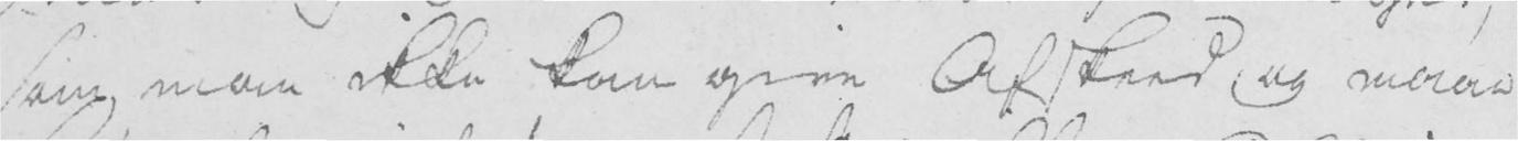som man ikke kan give afskeed og maae
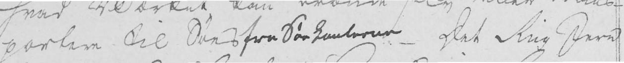portere til Søes fra Søekanterne. - Det Rug Jern
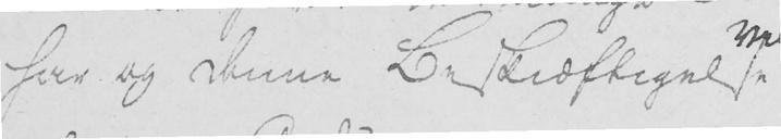har og denne Beskiæftigelse
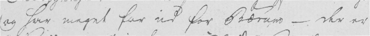og har meget for ud for Bærum - der er
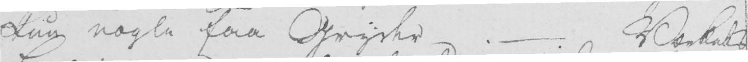kun nogle paa Gryder. - Værkets
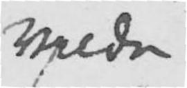vilde
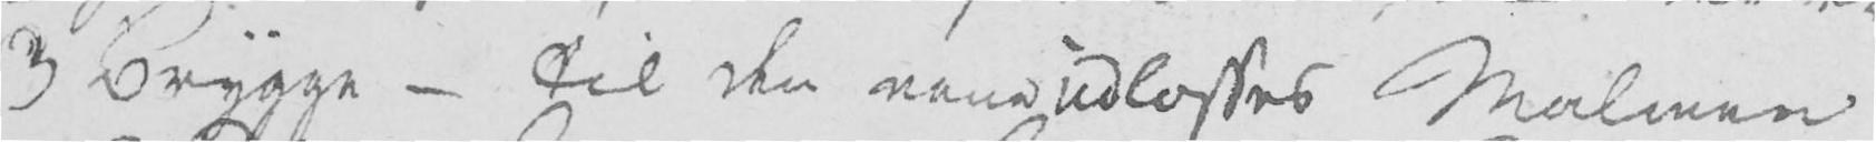3 Brygge - til den eene udlosses Malmen
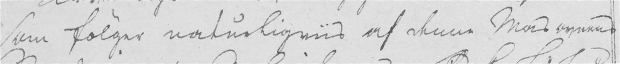som følger naturligviis af denne Masovnens
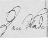Den Skade
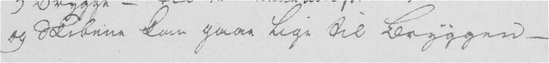og Skibene kan gaae lige til Bryggen -
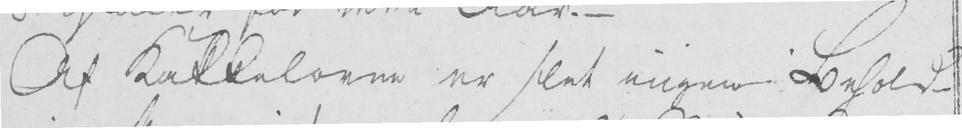Af Kakkelovne er slet ingen Behold-
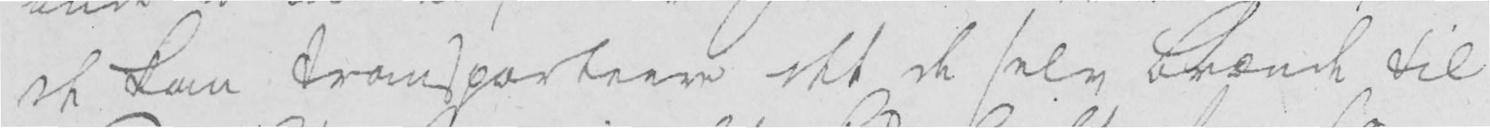de kan transporteere det de selv brænde til
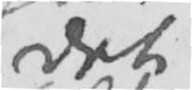det
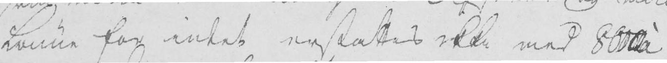lønne for intet erstattes ikke med 8000 à
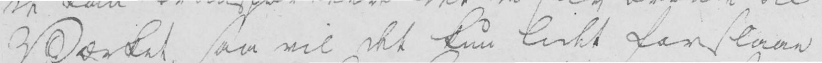Værket, saa vil det kun lidet forslaae
